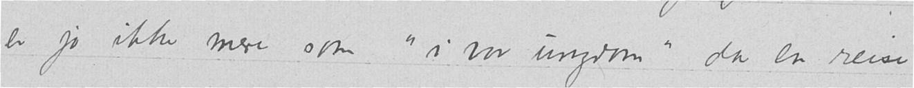er jo ikke mere som "i vor ungdom" da en reise
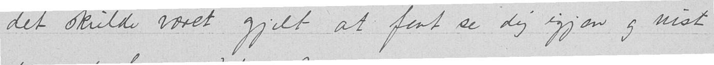det skulde været gjilt at faat se dig igjen og vist
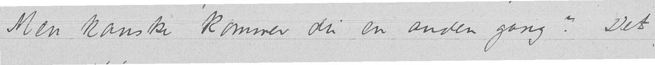Men kanske kommer du en anden gang? Det
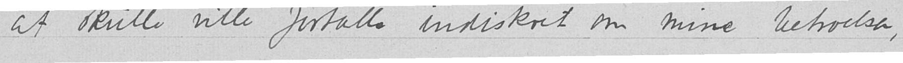at skulle ville fortælle indiskret om mine betroelser,
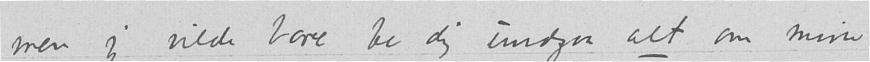men jeg vilde bare be dig undgaa alt om mine
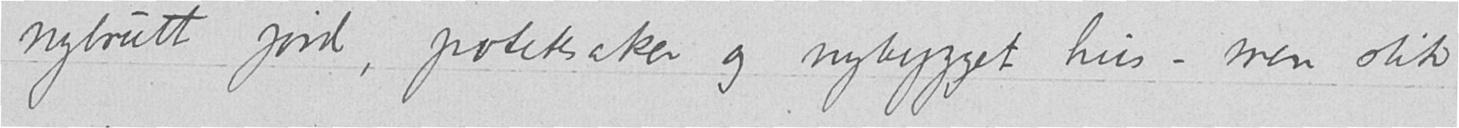nybrutt jord, potetesaker og nybygget hus — men slik
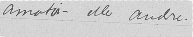amatør- eller andre.
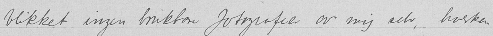blikket ingen brukbare fotografier av mig selv, hverken
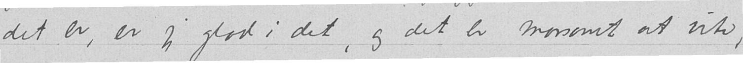det er, er jeg glad i det, og det er morsomt at vite,
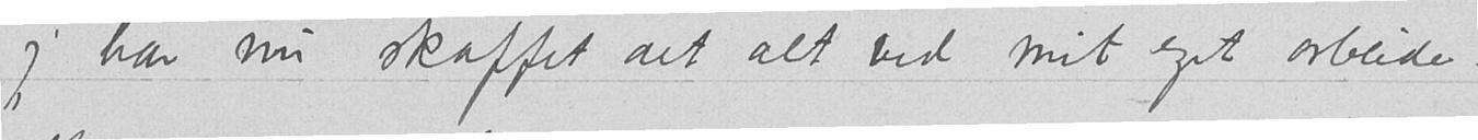jeg har nu skaffet det alt ved mit eget arbeide.
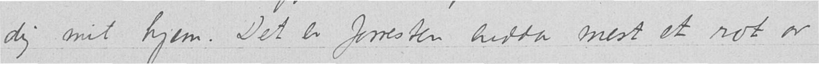dig mit hjem. Det er forresten endda mest et rot av
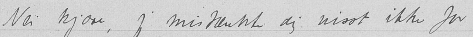Nei kjære, jeg mistenkte dig visst ikke for
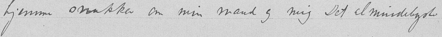hjemme snakker om min mand og mig. Det almindeligste
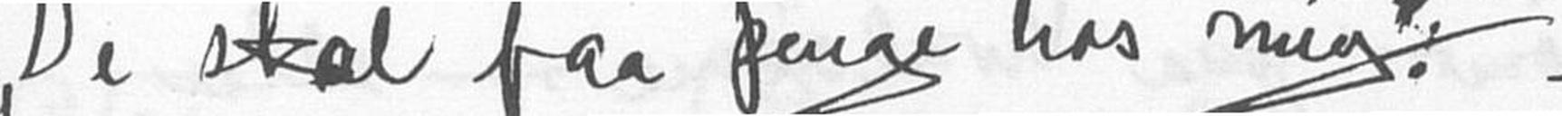"De skal faa penge hos mig!"
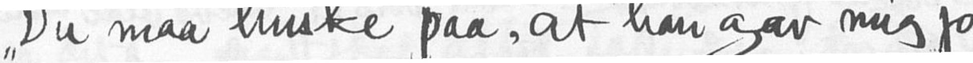"Du maa huske paa, at han gav mig jo
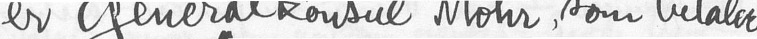er generalkonsul Mohr, som betaler
